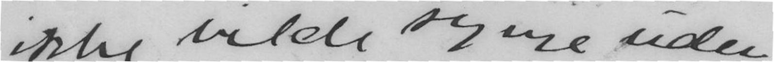ikke vilde synge uden
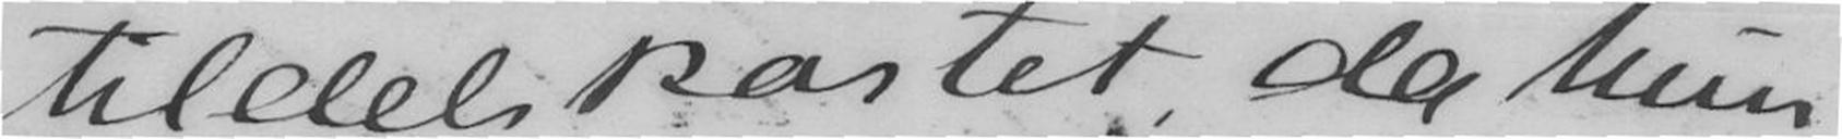tildels kastet da hun
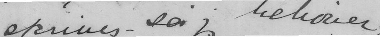skrives så jeg behøver
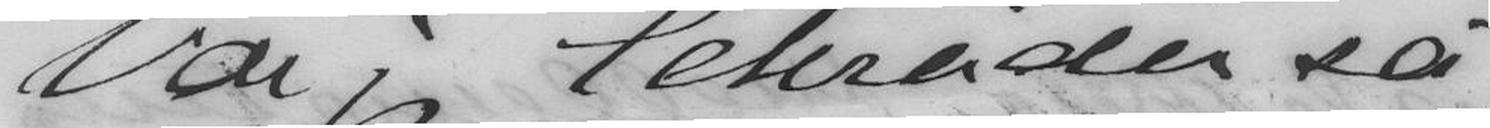var jeg Schrøder så
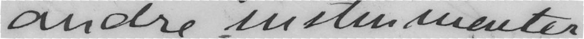andre instrumenter.
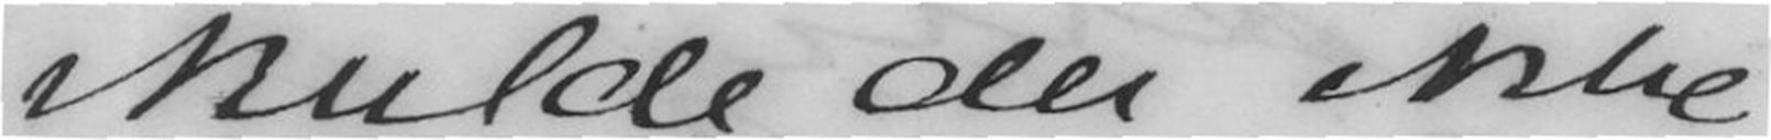skulde der ikke
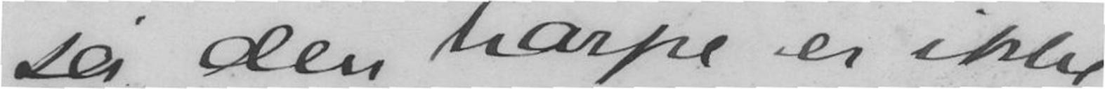- så den harpe er ikke
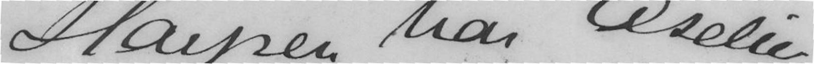Harpen har Oselio
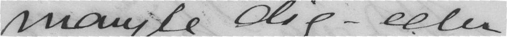mangle dig - eller
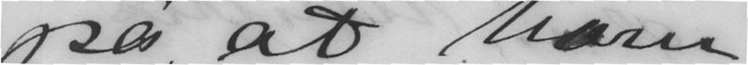på, at ham
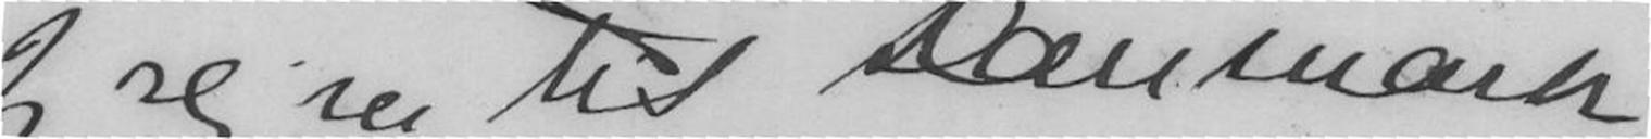Jeg rejser til Danmark
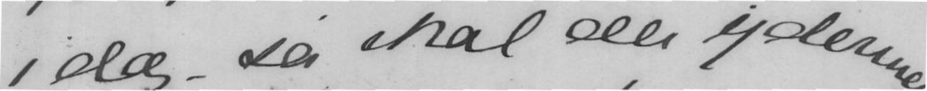i dag - så skal der ejdermen
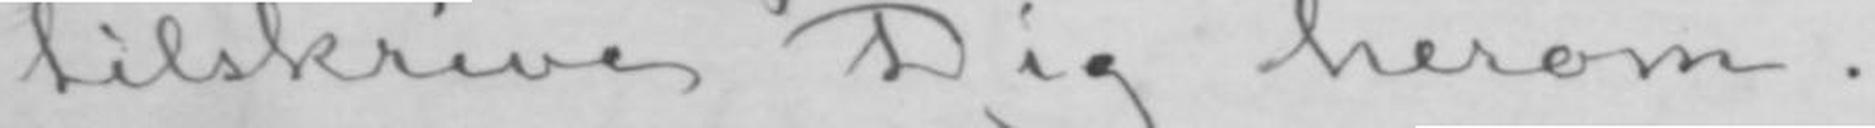tilskrive Dig herom.
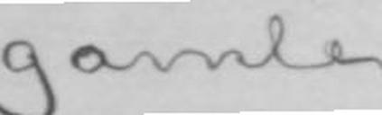gamle.
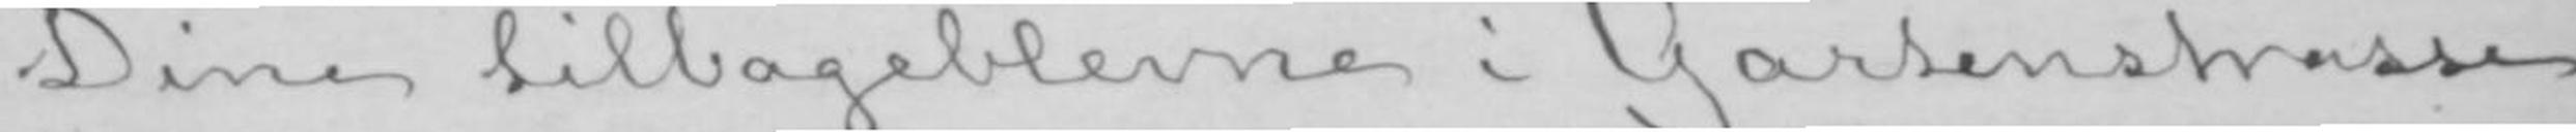Dine tilbageblevne i Gartenstrasse
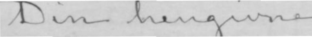Din hengivne
